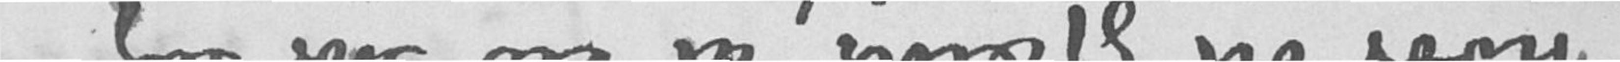hvor det gjælder at en stor sag
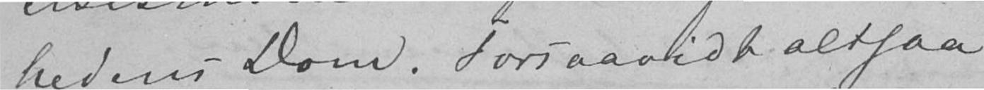hedens Dom. Forsaavidt altsaa
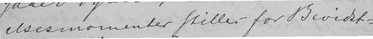elsesmomenter stilles for Bevidst-
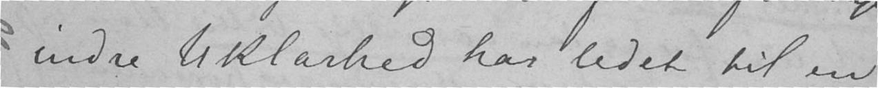indre Uklarhed har ledet til en
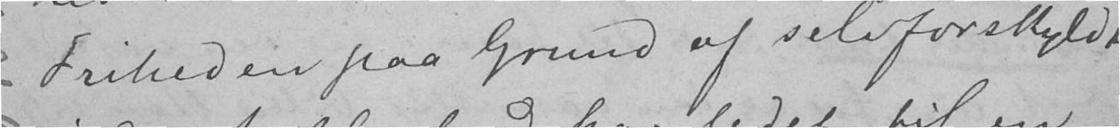Friheden paa Grund af selvforskyldt
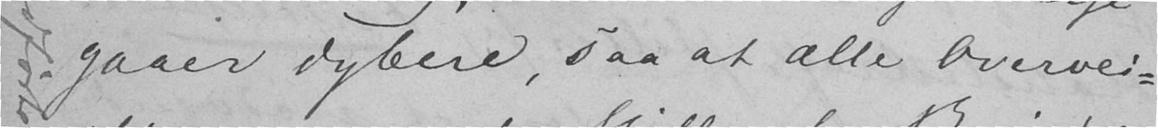gaaer dybere, saa at alle Overvei-
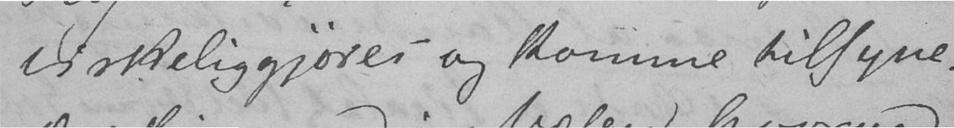virkeliggjøres og komme tilsyne.
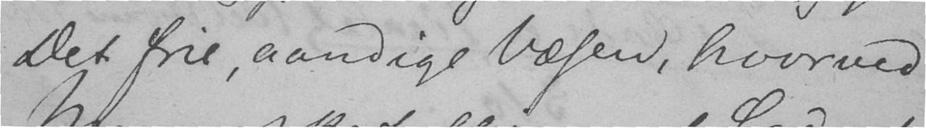Det frie, aandige Væsen, hvorved
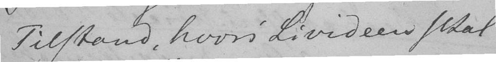Tilstand, hvori Livideen skal
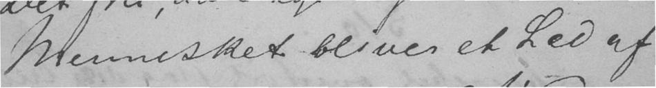Mennesket bliver et Led af
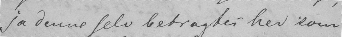ja denne selv betragtes her som
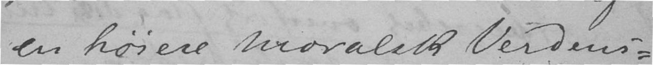en høiere moralsk Verdens-
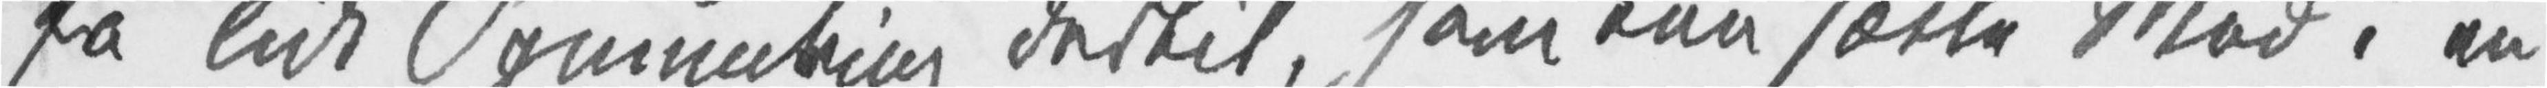få lidt Opmuntring dertil, som kan sætte Mod i en
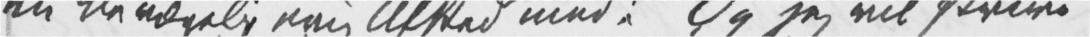en bevægelig evig Afsked med! Og jeg vil skrive
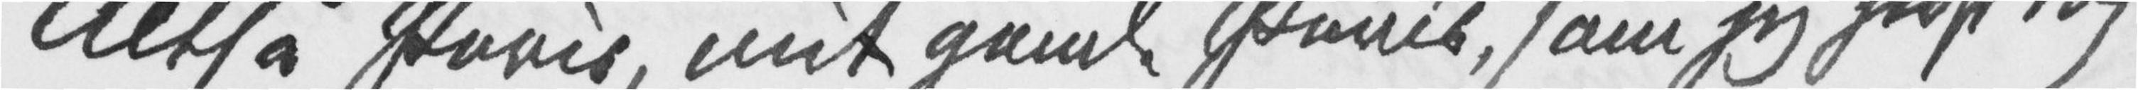Altså Paris, mit gamle Paris, som jeg sidst tog
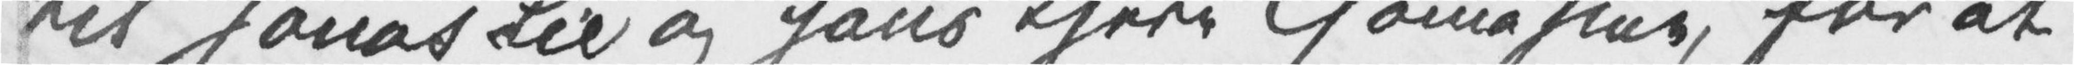til Jonas Lie og hans kjere Thomasine, for at
